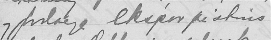og forlange eksporpiations
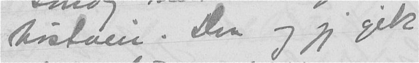høstveir. Don og jeg gik
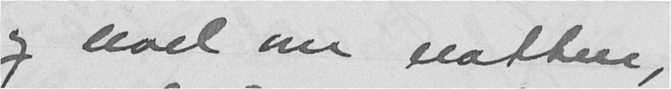og uvel om natten,
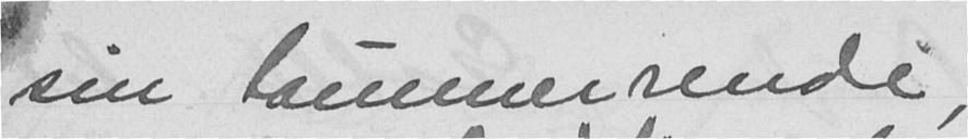sin tømmerrende,
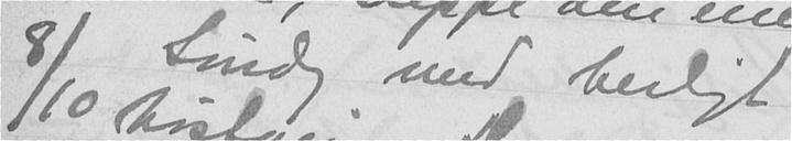8/10 Søndag med herligt
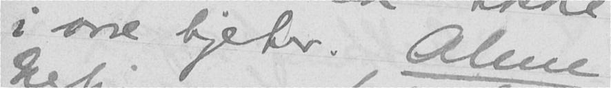i vore hjerter. Alene
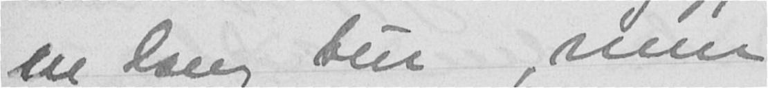en lang tur, men
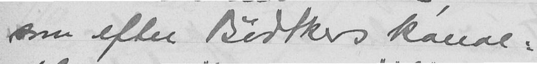som efter Bødtkers kanal-
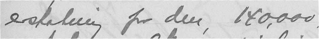erstatning for den, 140,000,
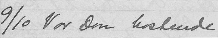9/10 Var Don hostende
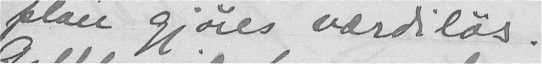plan gjøres værdiløs.
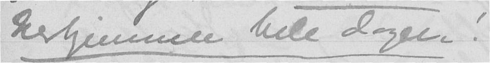herhjemme hele dagen!
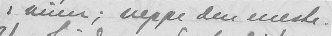i veien; neppe den eneste.
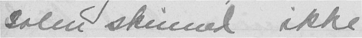solen skinned ikke
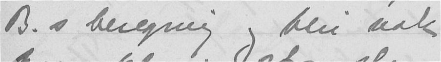B. s beregning og blir nok
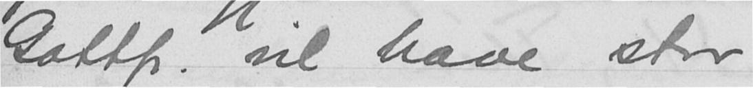Gottfr. vil have stor
